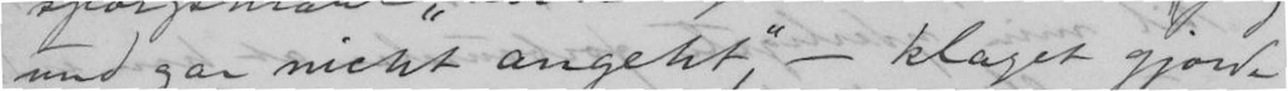und gar nicht angeht", - klaget gjorde
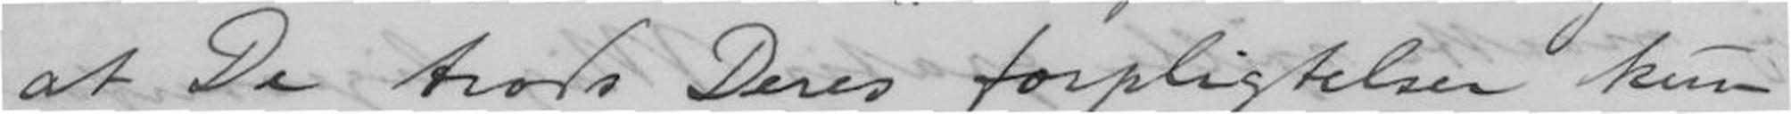at De trods Deres forpligtelse kun
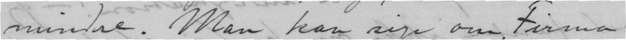mindre. Man kan sige om "Firma
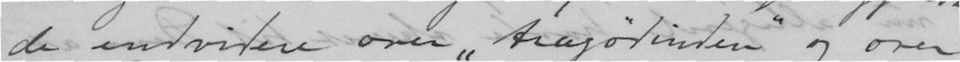de endvidere over "tragödinden" og over
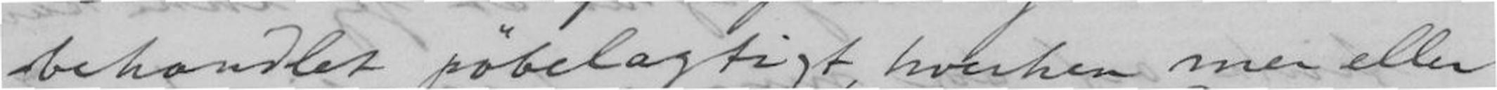behandlet pøbelagtigt, hverken mer eller
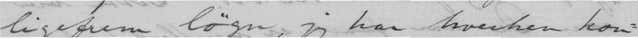ligefrem løgn, jeg har hverken kon-
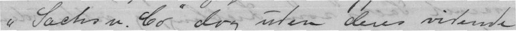"Sachs u. Co" dog uden deres vidende
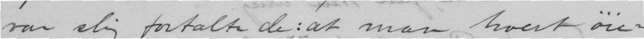var slig fortalte de: at man hvert øie-
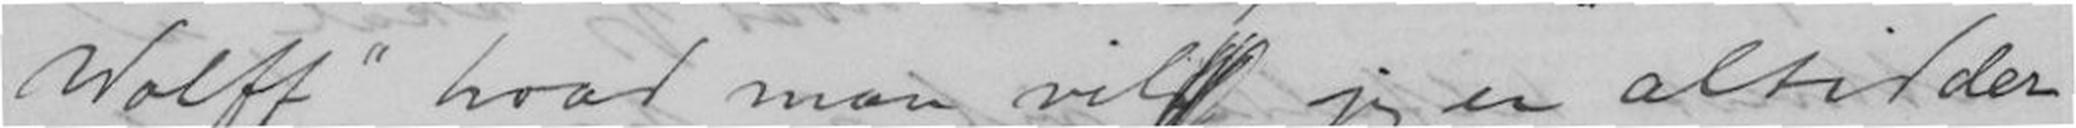Wolff" hvad man vil, jeg er altid der
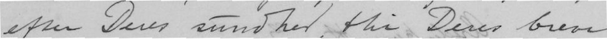efter Deres sundhed, thi Deres breve
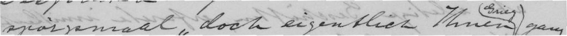spørgsmaal "doch eigentlich Ihnen ganz
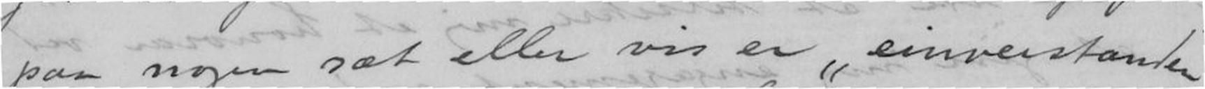paa nogen sæt eller vis er "einverstanden"
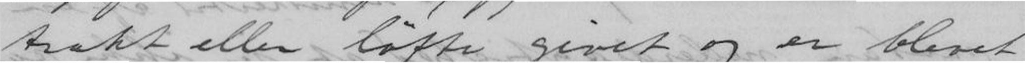trakt eller løfte givet og er blevet
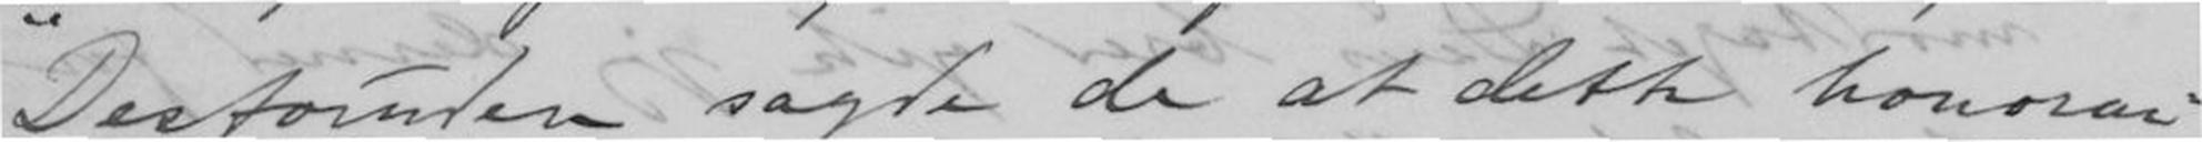Desforuden sagde de at dette honorar-
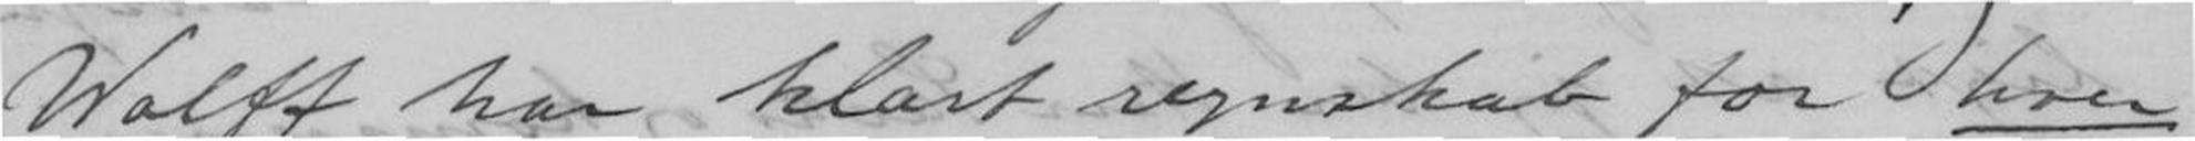Wolff har klart regnskab for hver
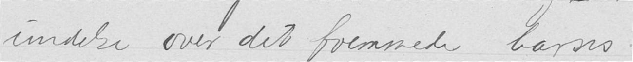undelse over det fremmede barns
